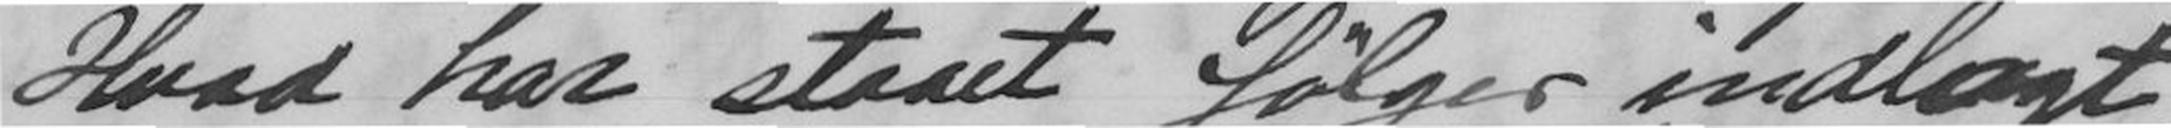Hvad har staaet følger indlagt.
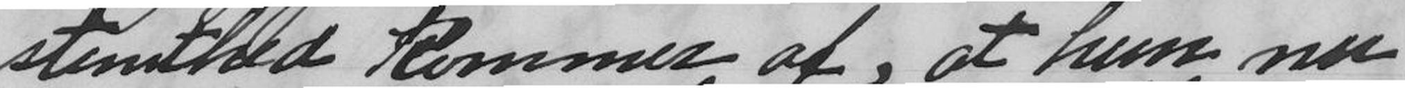stemthed kommer af, at hun nu
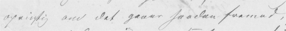oprigtig om det gaaer saadan fremad,
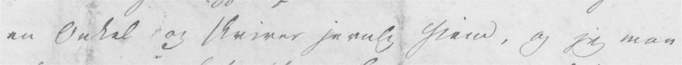en Onkel og skriver jevnlig hjem, og jeg maa
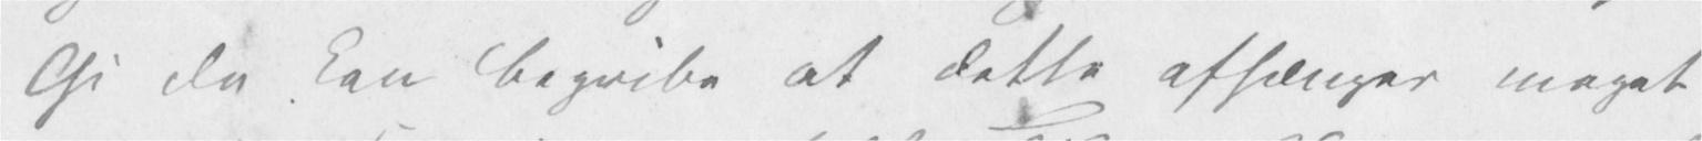Thi Du kan begribe at dette afhænger meget
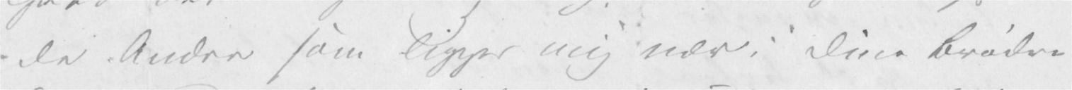de Andre som ligger mig nær: Dine Brødre
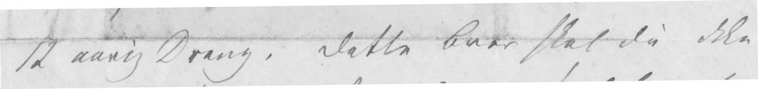12 aarig Dreng. Dette Brev skal Du ikke
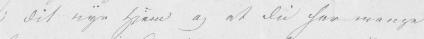i Dit nye Hjem og at Du har mange
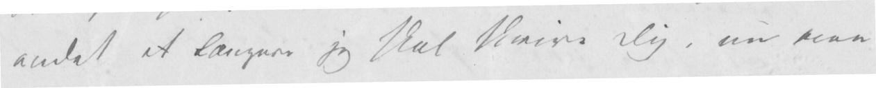andet et Længere jeg skal skrive Dig, nu maa
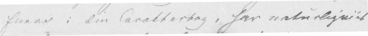Enere i Din Carakterbog, har naturligviis
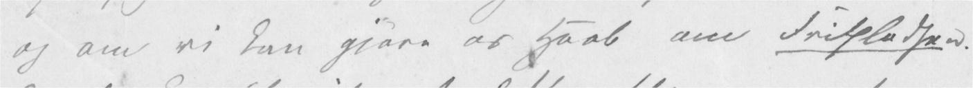og om vi kan gjøre os Haab om Fripladsen.
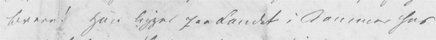Breve! Han ligger paa Landet i Sommer hos
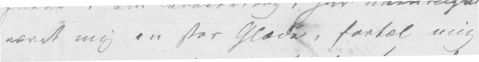været mig en stor Glæde, fortæl mig
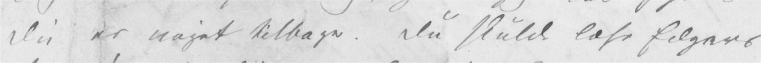Du er noget tilbage. Du skulde læse Edgars
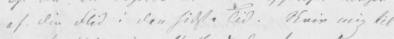af Din Flid i den sidste Tid. Skriv mig til
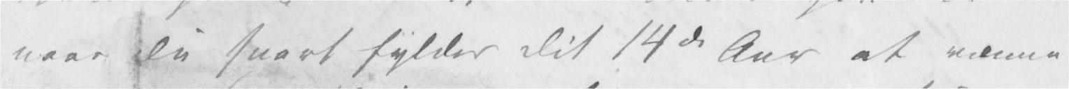naar Du snart fylder Dit 14de Aar at vænne
